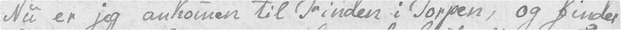Nu er jeg ankommen til Finden i Torpen, og finder
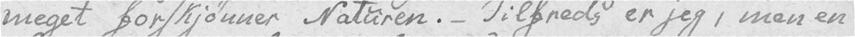meget forskjønner Naturen. – Tilfreds er jeg, men en
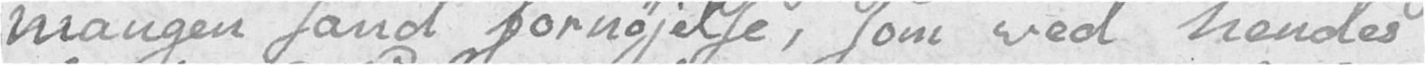mangen sand fornøjelse, som ved hendes
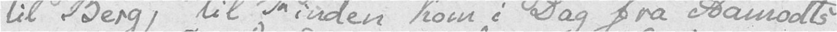til Berg, til Finden kom i Dag fra Aamodts
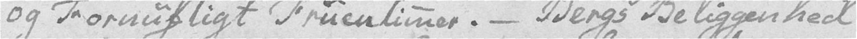og Fornuftigt fruentimmer. – Bergs Beliggenhed
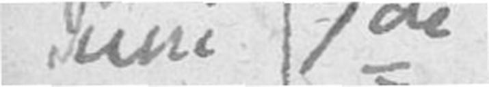Juni 7de
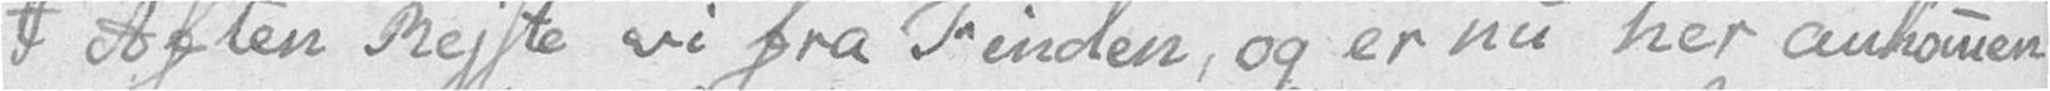I Aften Rejste vi fra Finden, og er nu her ankommen
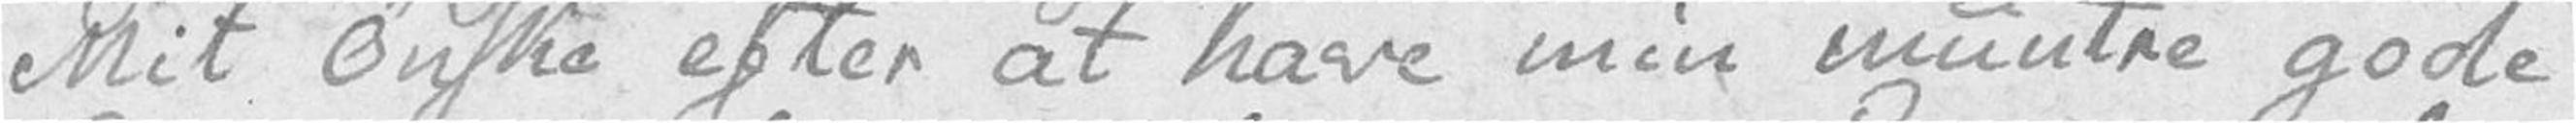Mit Ønske efter at have min muntre gode
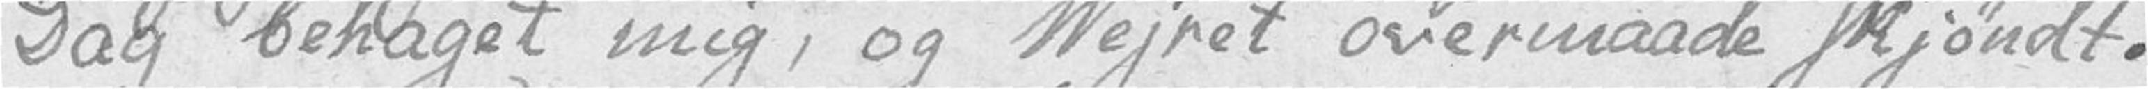Dag behaget mig, og Vejret overmaade skjøndt.
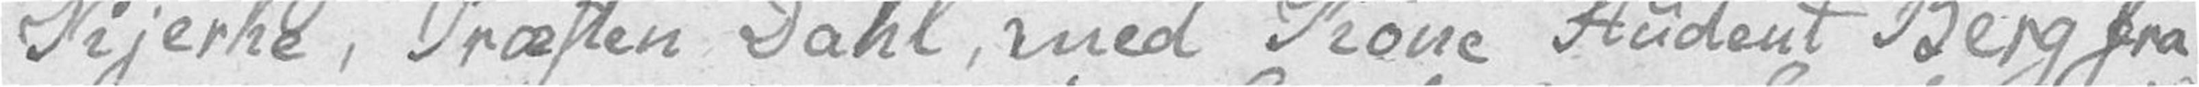Kjerke, Præsten Dahl, med Kone Student Berg fra
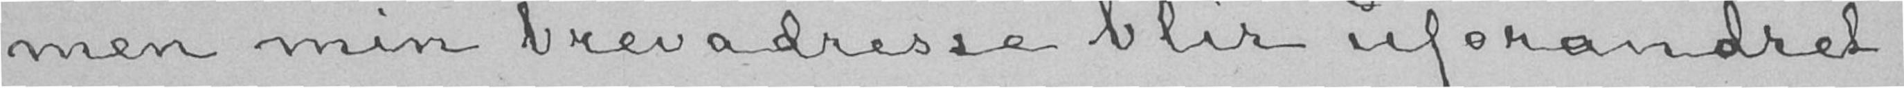men min brevadresse blir uforandret.
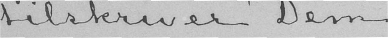tilskriver Dem.
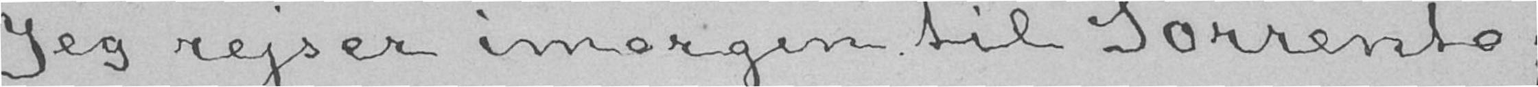Jeg rejser imorgen til Sorrento;
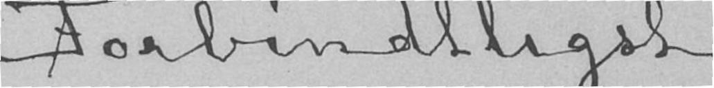Forbindtligst
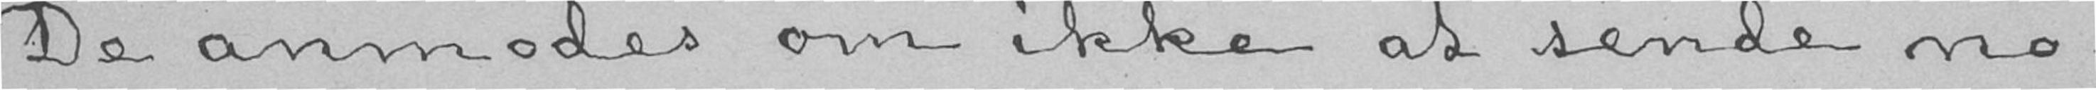De anmodes om ikke at sende no-
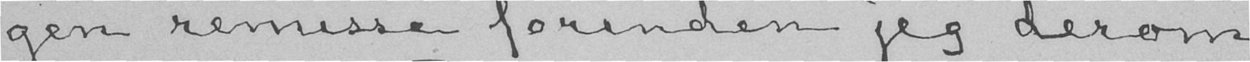gen remisse forinden jeg derom
